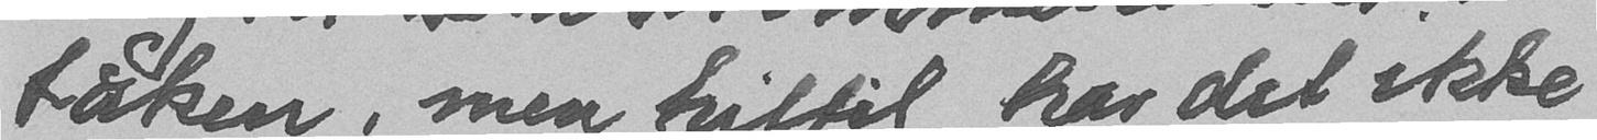tåken, men hittil har det ikke
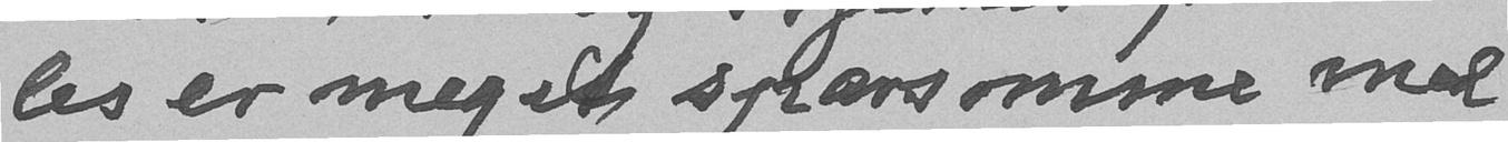les er meget sparsomme med
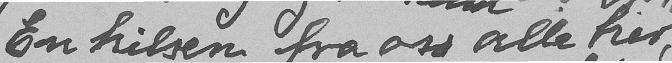En hilsen fra oss alle her,
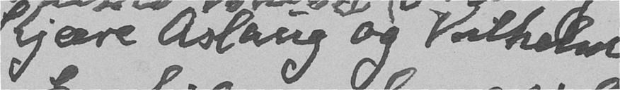Kjære Aslaug og Vilhelm
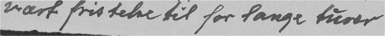vært fristelse til for lange turer
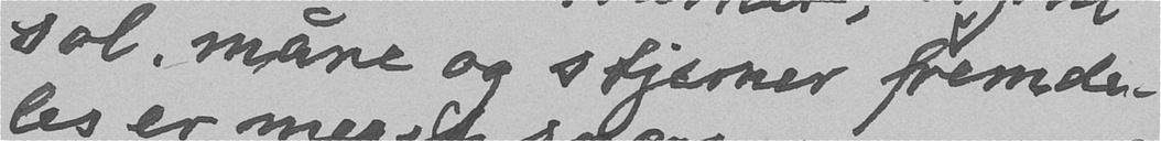sol, måne og stjerner fremde-
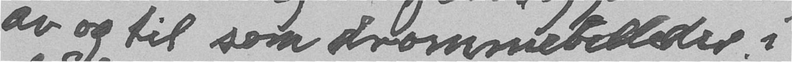av og til som drømmebilleder i
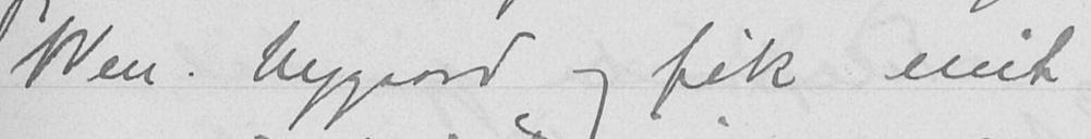Wen. Nygaard og fik mit
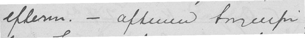efterm. - aftenen Sorgenfri,
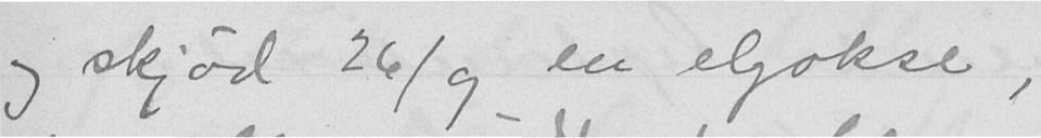og skjød 26/9 en elgokse,
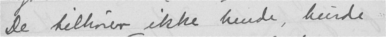De tilhører ikke hende, burde
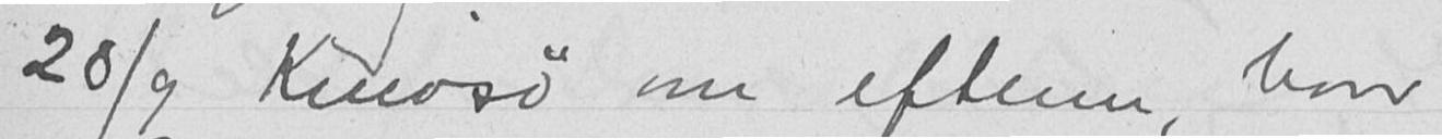20/9 Knivsø om efterm, hvor
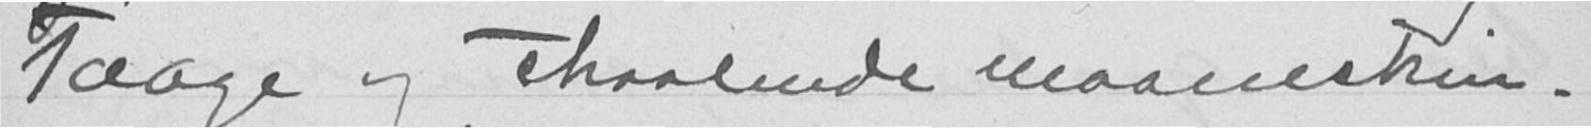Taage og straalende maaneskin.
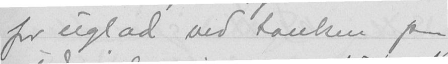for uglad ved tanken paa
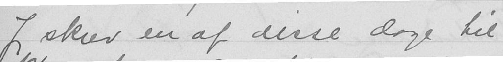Jeg skrev en af disse dage til
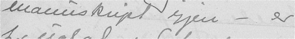manuskript igjen - er
